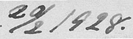20/2 1928.
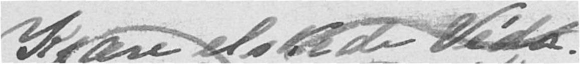Kjære elskede Veda
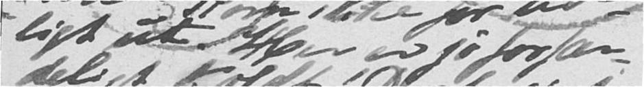ligt ut. Her er jo forfær-
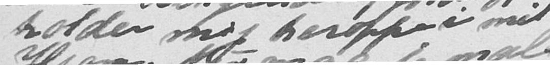holder mig heroppe i mit
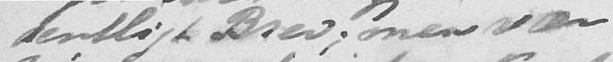dentligt Brev; men væn
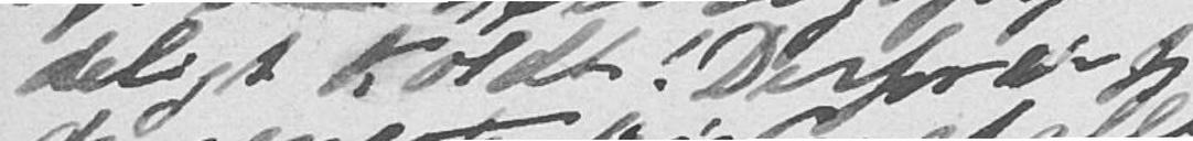deligt Koldt. Derfor er jeg
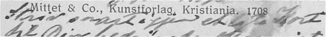Skriv snart igjen et lite Kort
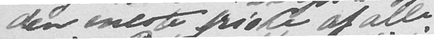den eneste friske af alle
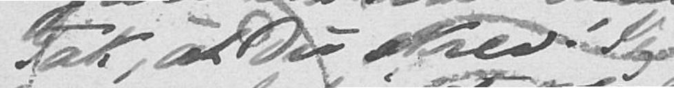Tak, at Du skrev. Jeg
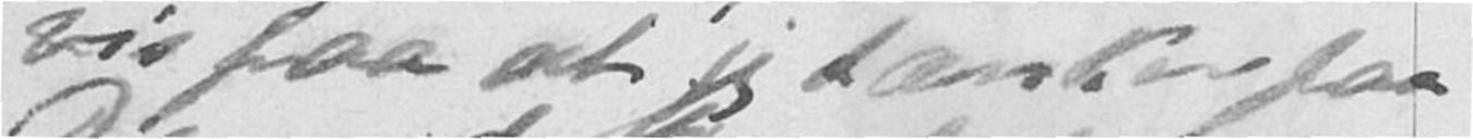vis paa at jeg tænker faa
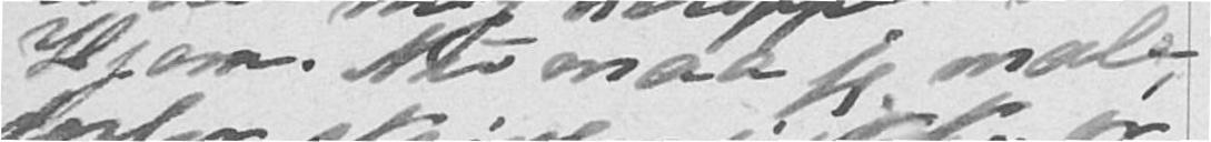Hjem. Nu maa jeg male,
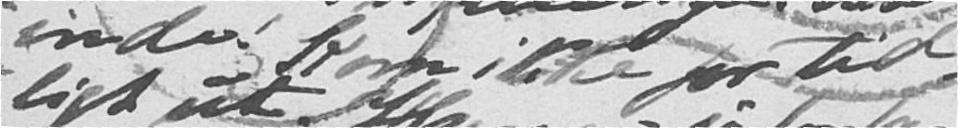inde! Kom ikke for tid-
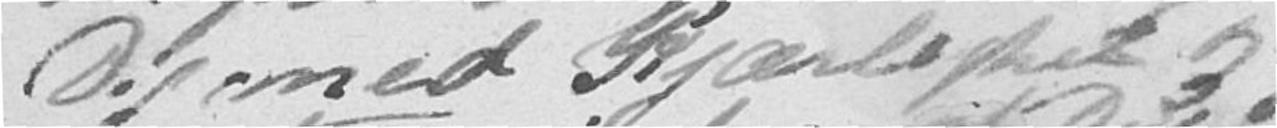Dig med Kjærlighet og
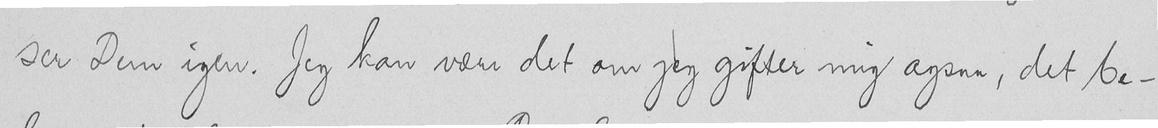ser Dem igen. Jeg kan være det om jeg gifter mig ogsaa, det be-
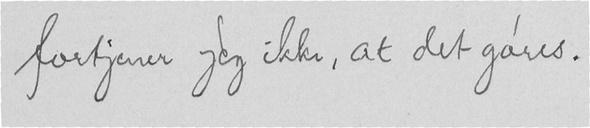fortjener jeg ikke, at det gøres.
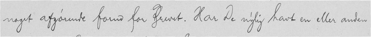noget afgørende forud for Brevet. Har De nylig havt en eller anden
